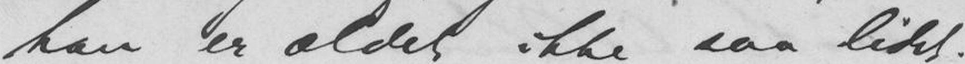han er ældet ikke saa lidet.
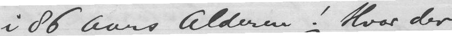i 86 Aars Alderen! Hvor der
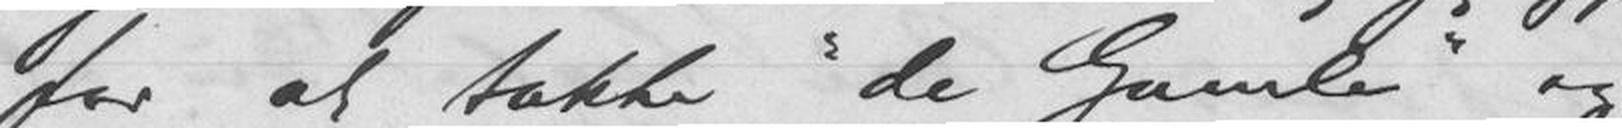for at takke "de Gamle" og
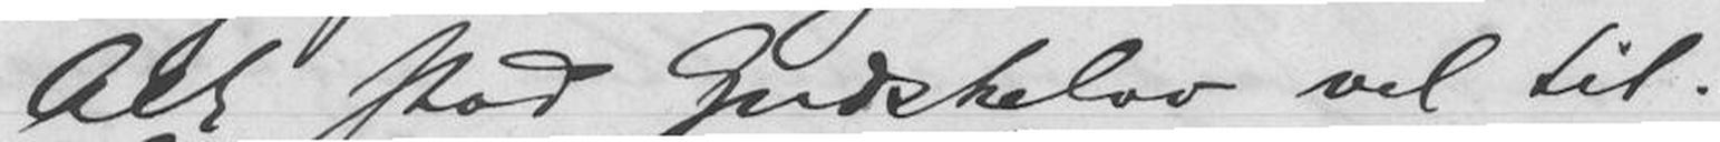Alt stod Gudskelov vel til.
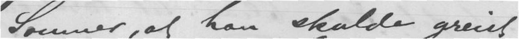Sommer, at han skulde greiet
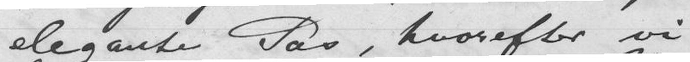elegante Pas, hvorefter vi
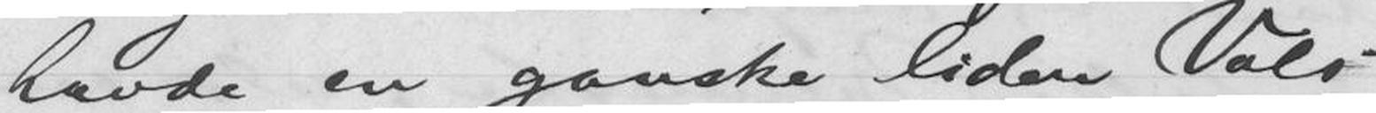havde en ganske liden Vals
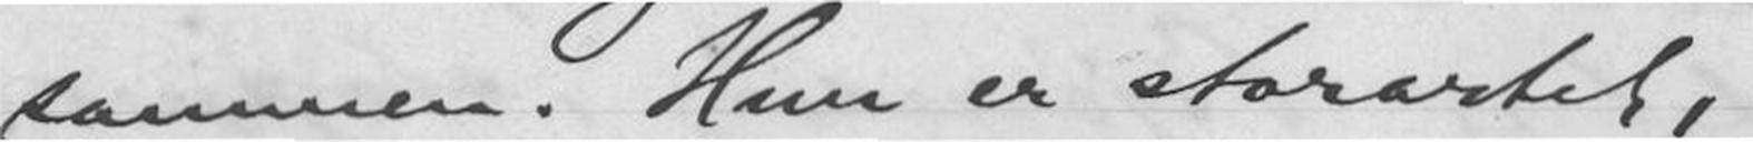sammen. Hun er storartet, -
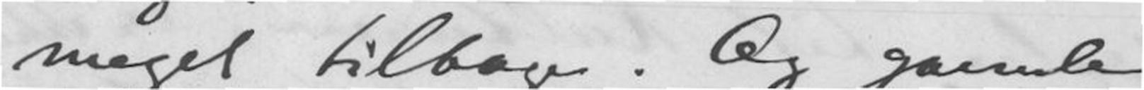meget tilbage. Og gamle
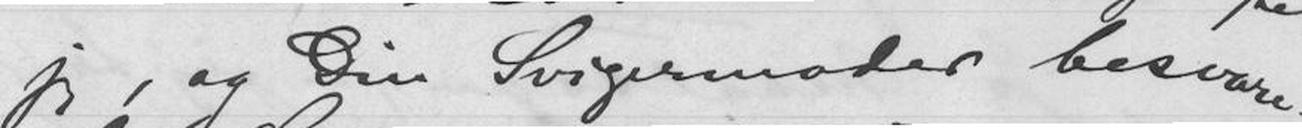jeg, og Din Svigermoder besvare-
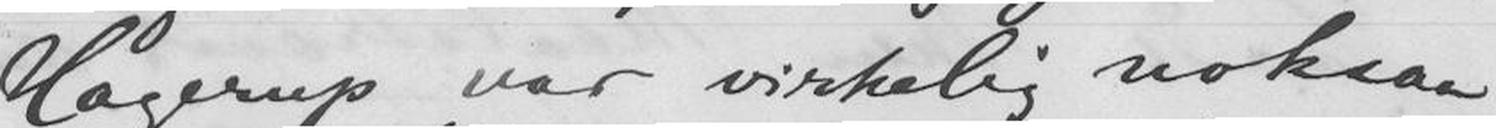Hagerup var virkelig noksaa
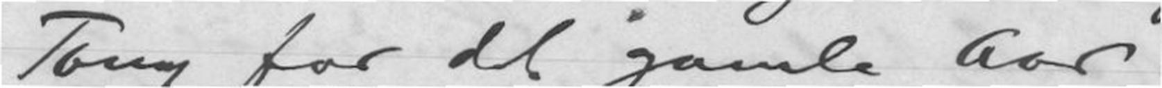Tony for det gamle Aar.
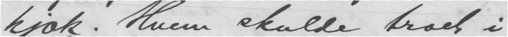kjæk. Hvem skulde troet i
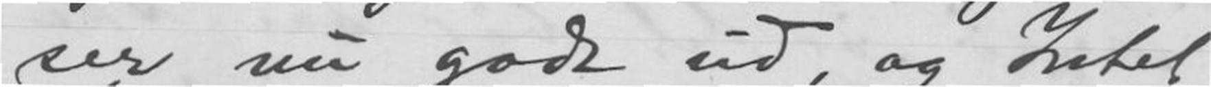ser nu godt ud, og Intet
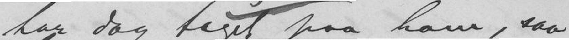har dog taget paa ham, saa
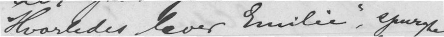"Hvorledes lever Emilie" spurgte
